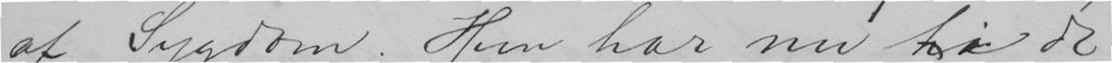af Sygdom. Hun har nu i de
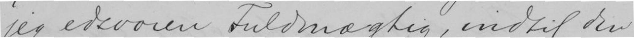jeg edsvoren Fuldmægtig, indtil den
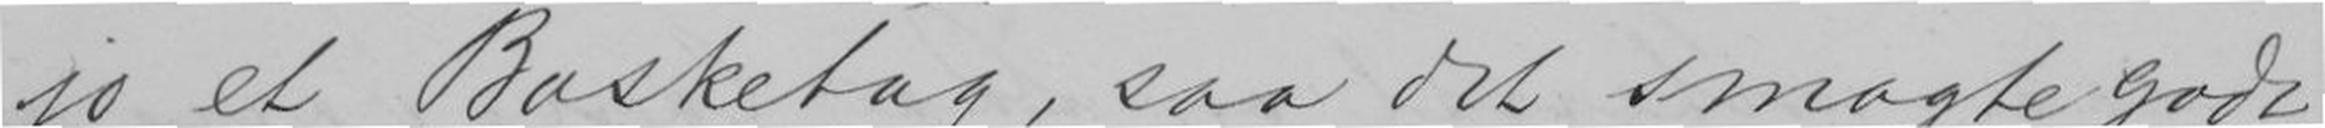jo et Basketag, saa det smagte godt
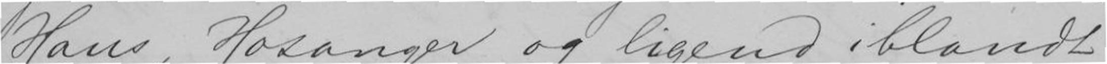Haus, Hosanger og ligeud iblandt
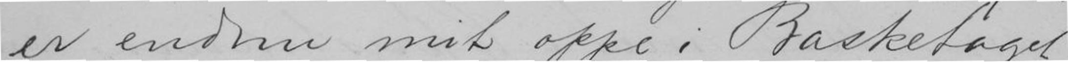er endnu mit oppe i Basketaget
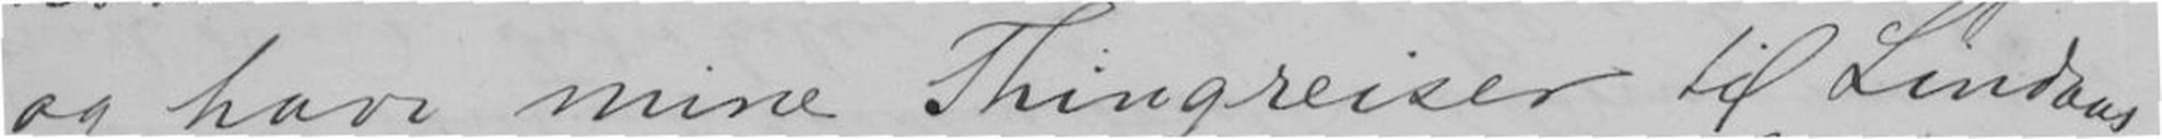og have mine Thingreiser til Lindaas,
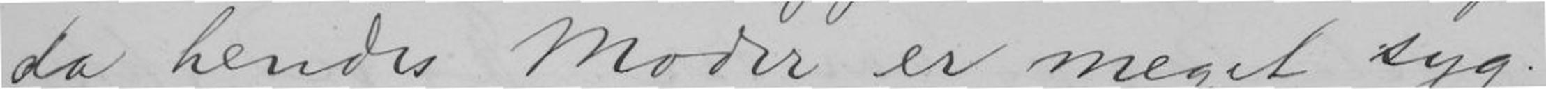da hendes Moder er meget syg.
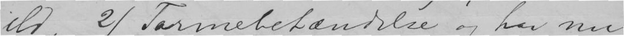ild, 2) Tarmebetændelse og har nu
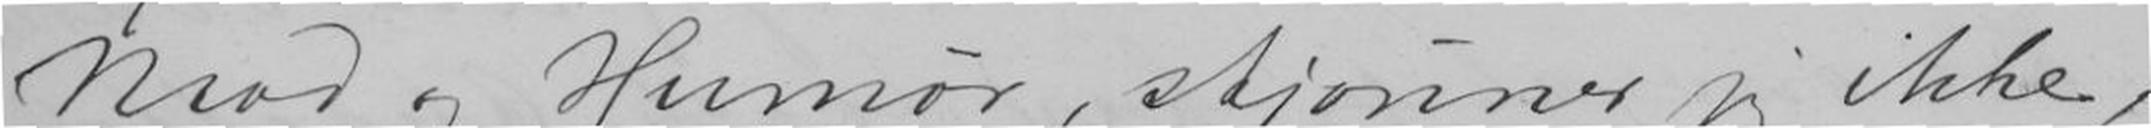Mod og Humør, skjønner jeg ikke,
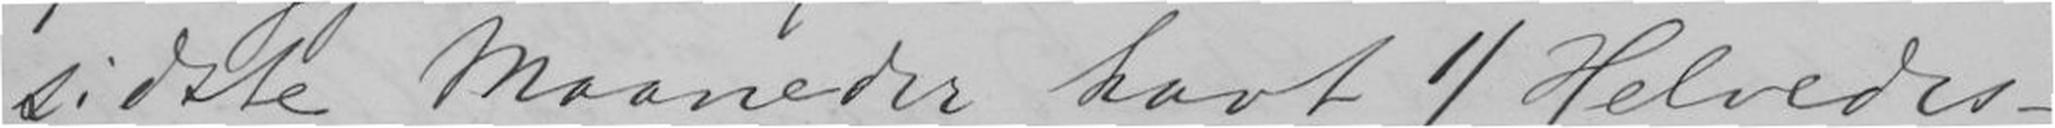sidste Maaneder havt 1) Helvedes-
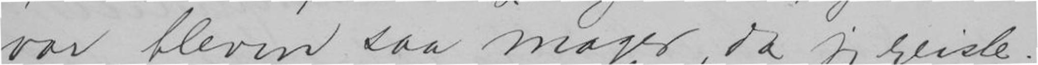var bleven saa mager, da jeg reiste.
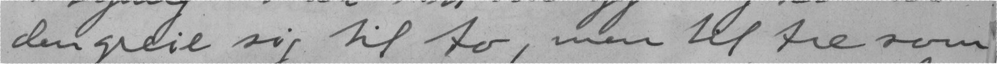den greie sig til to, men til tre som
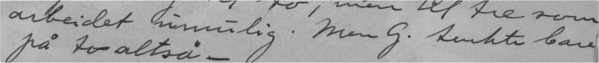arbeidet umulig. Men G. tenkte bare
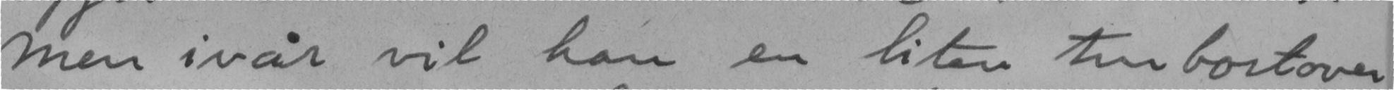Men i vår vil han en liten tur bortover
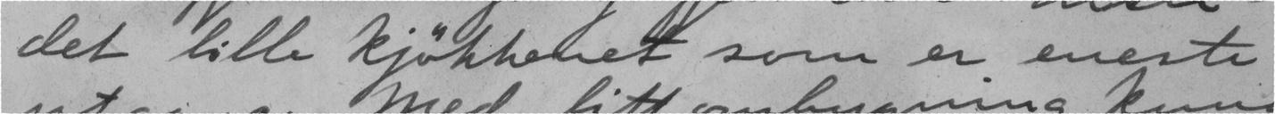det lille kjøkkenet som er eneste
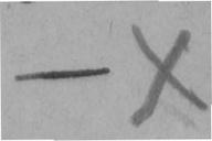- x
